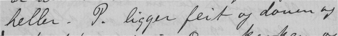heller. P. ligger feit og doven og
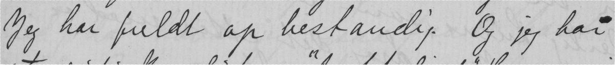Jeg har fuldt op bestandig. Og jeg har
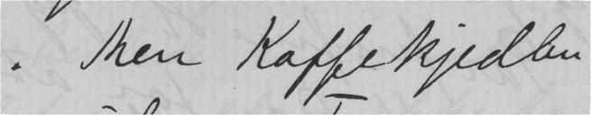. Men Kaffekjedlen
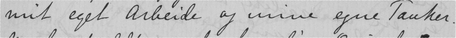mit eget arbeide og mine egne Tanker.
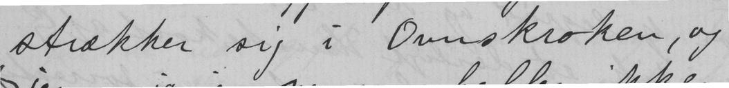strækker sig i Ovnskroken, og
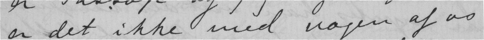er det ikke med nogen af os
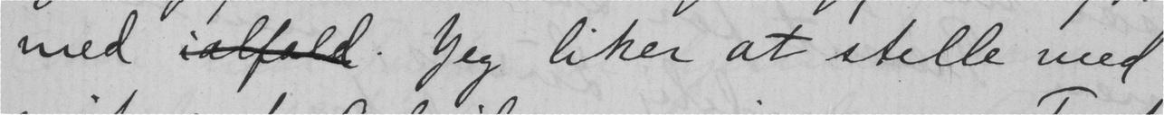med ialfald. Jeg liker at stelle med
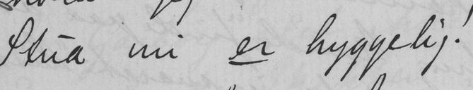Stua mi er hyggelig!
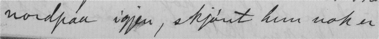nordpaa igjen, skjønt hun nok er
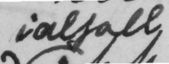ialfall
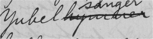jubelhymner
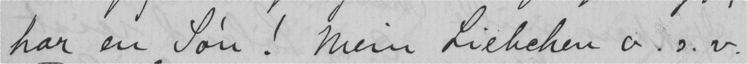har en Søn! Mein Liebchen o.s.v.
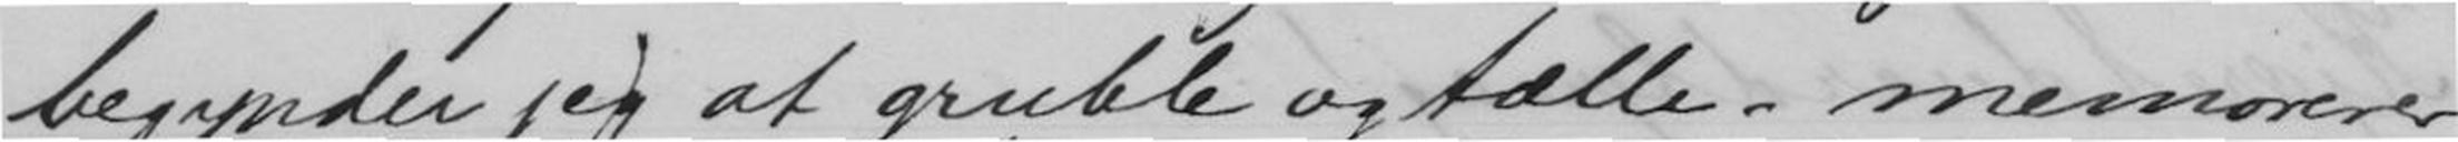begynder jeg at gruble og tælle, memorerer
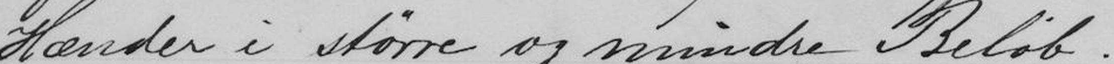Hænder i større og mindre Beløb.
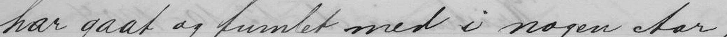har gaat og tumlet med i nogen Aar,
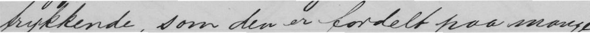trykkende, som den er fordelt paa mange
